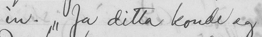in. "Ja ditta konde eg
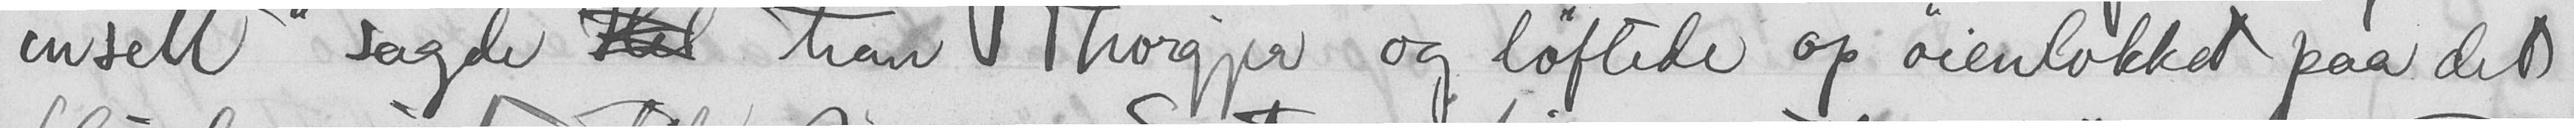insett" sagde  han Thorgjer og løftede op øienlokket paa det
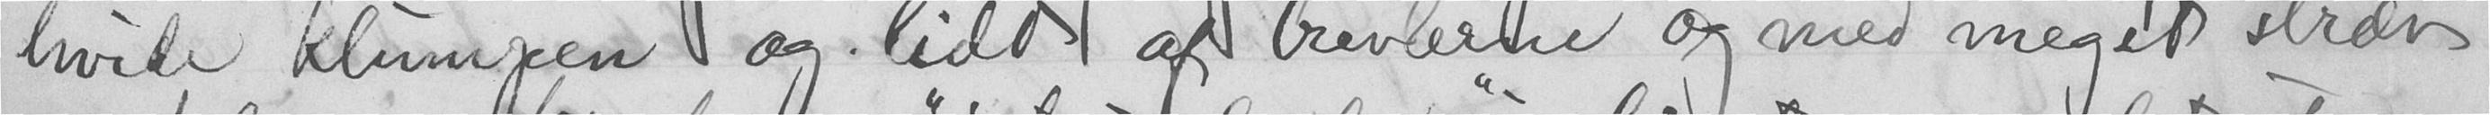hvide klumpen og lidt af trevlerne og med meget stræv
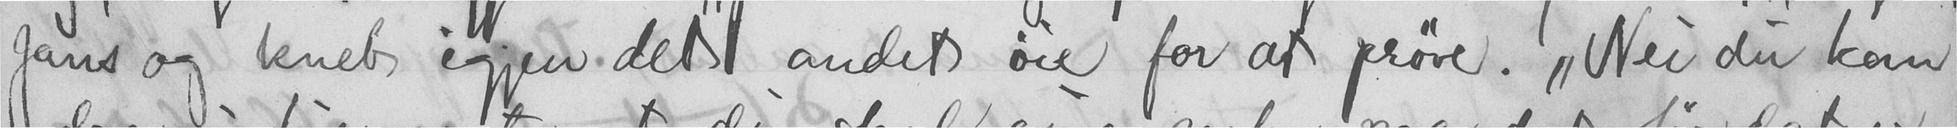Jans og kneb igjen det andet øie for at prøve. "Nei du kan
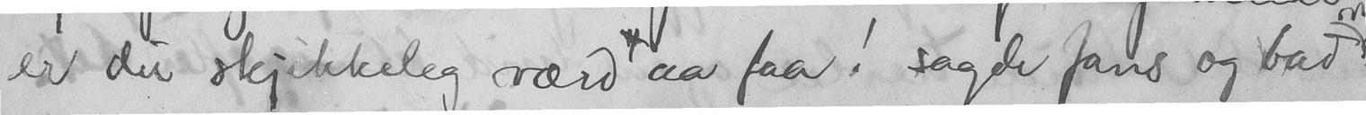er du skjkkelig værd aa faa! sagde Jans og bad
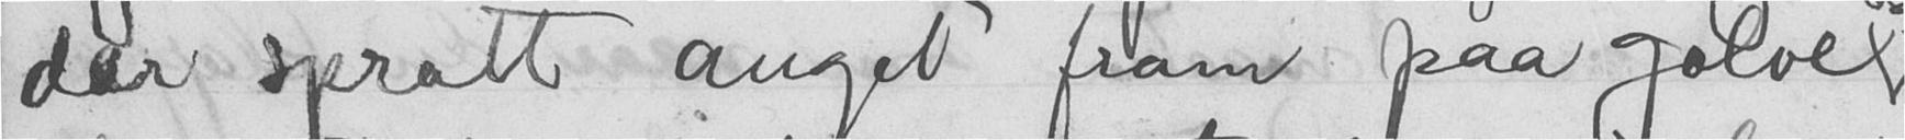der spratt auget fram paa golve
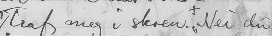traf meg i skoen. "Nei du
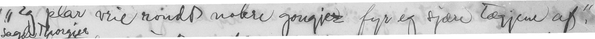"Eg plar vrie rondt nokre gongjer fyr eg sjære tægjene af".
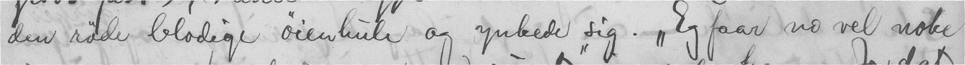den søde blodige øienhule og ynkede sig. "Eg faar no vel noke
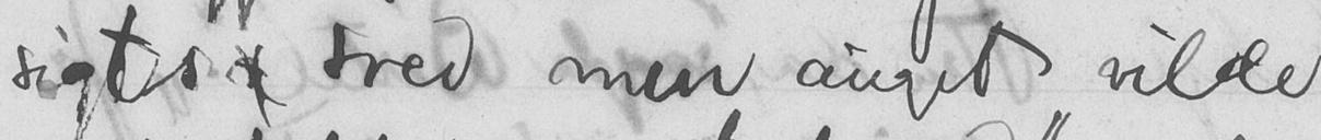sigts  vred men augets vilde
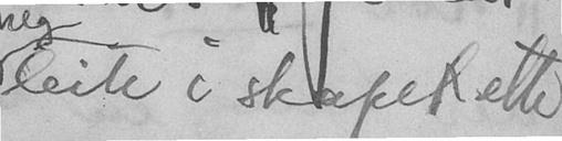leite i skapet ette
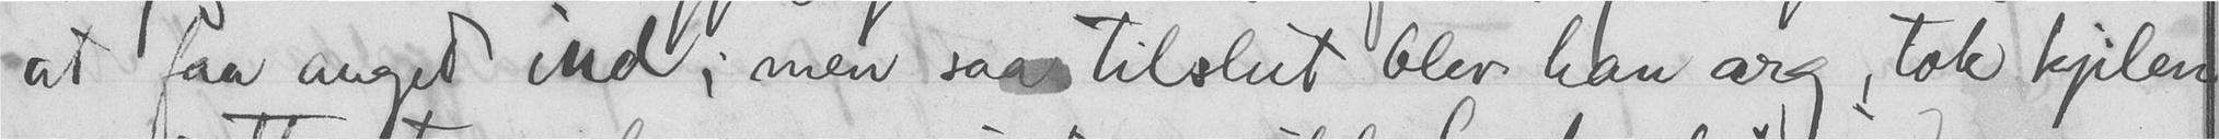at faa auget ind, men saa tilslut blev han arg, tok kjilen
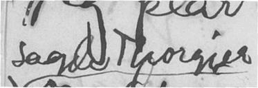sagde Thorgjer
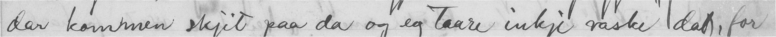dar kommen skjit paa da og eg taare inkje vaske dat, for
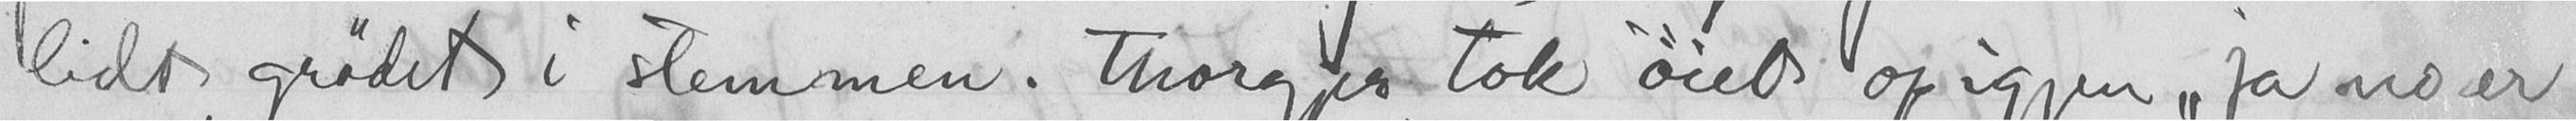lidt grødet i stemmen. Thorgjer tok øiet op igjen "ja no er
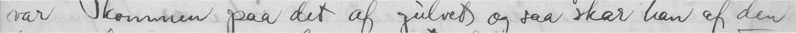var kommen paa det af gulvet og saa skar han af den
